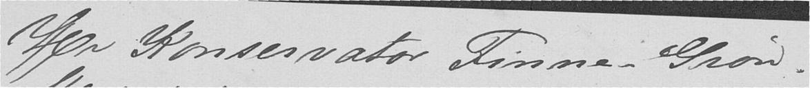Hr Konservator Finne-Grøn.
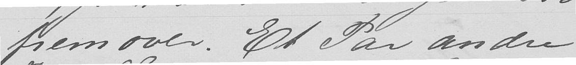fremover. Et Par andre
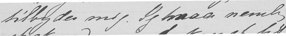tilbydes mig. Jeg maa nemlig
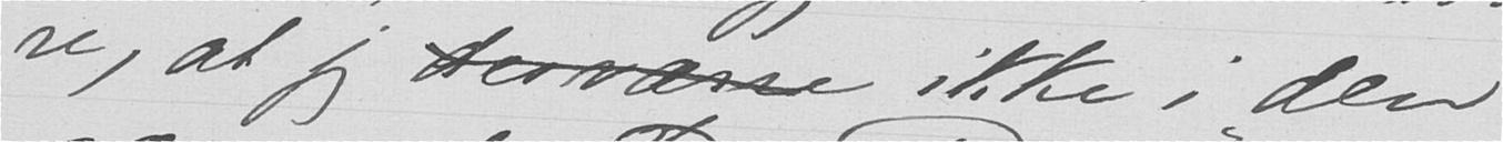re, at jeg desværre ikke i den
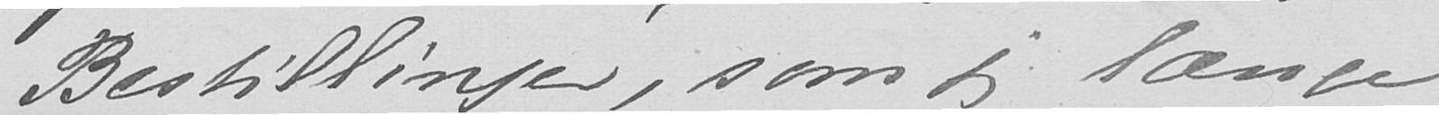Bestillinger, som jeg længe
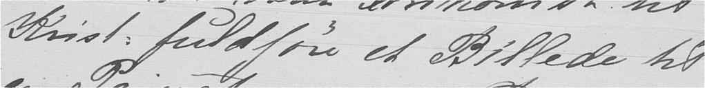Krist. fuldføre et Billede til
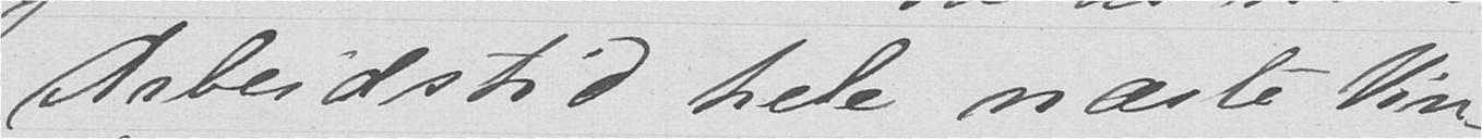Arbeidstid hele næste Vin-
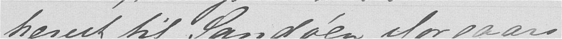herut til Sandøen iforgaars
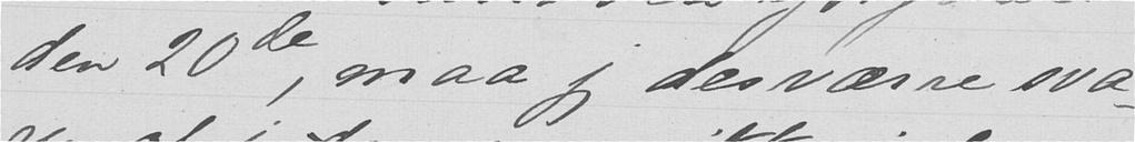den 20de, maa jeg desværre sva-
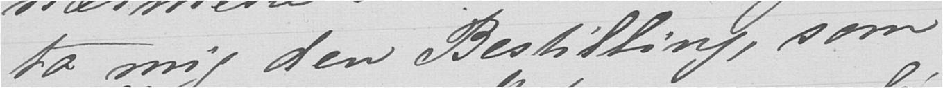ta mig den Bestilling, som
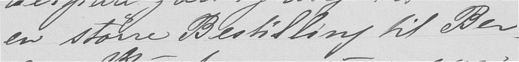en større Bestilling til Ber-
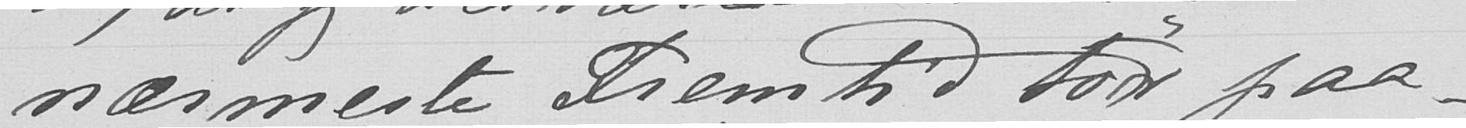nærmeste Fremtid tør paa-
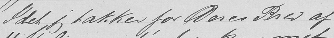Idet jeg takker for Deres Brev af
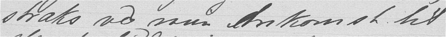straks ved min Ankomst til
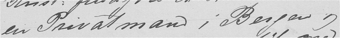en Privatmand i Bergen og
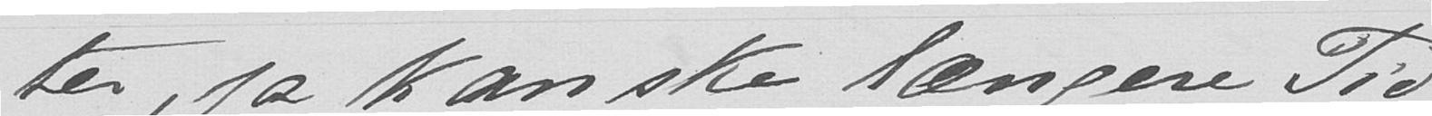ter, ja kanske længere Tid
